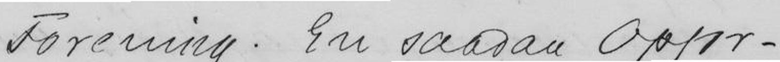Forening. En saadan Opfor-
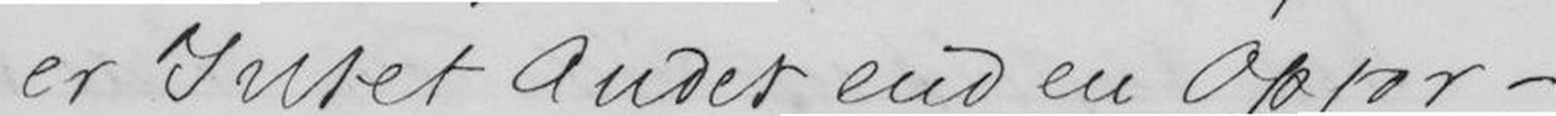er Intet Andet end en Opfor-
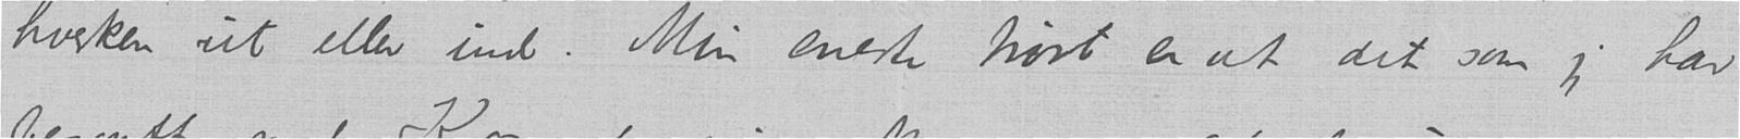hverken ut eller ind. Min eneste trøst er at det som jeg har
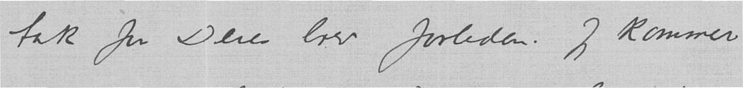tak for Deres brev forleden. Jeg kommer
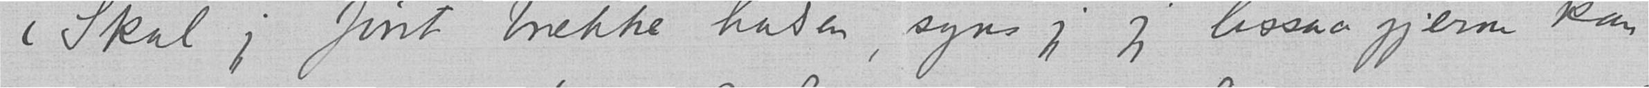(Skal jeg først brekke halsen, syns jeg jeg lissom gjerne kan
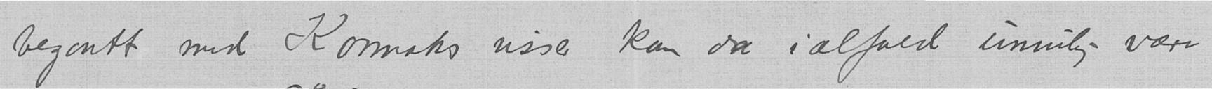begaatt med Kormaks viser kan da ialfald umulig være
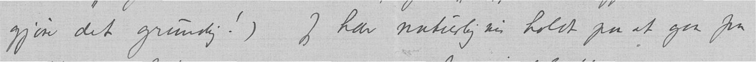gjøre det grundig!) Jeg har naturligvis holdt paa at gaa fra
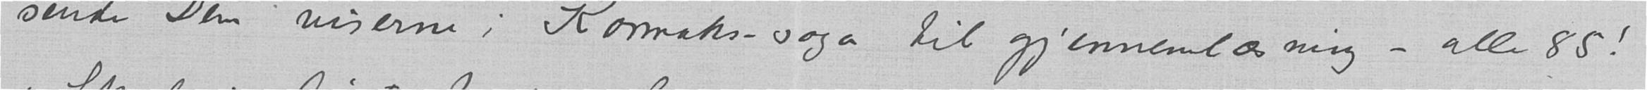sende Dem viserne i Kormaks-saga til gjennemlæsning – alle 85!
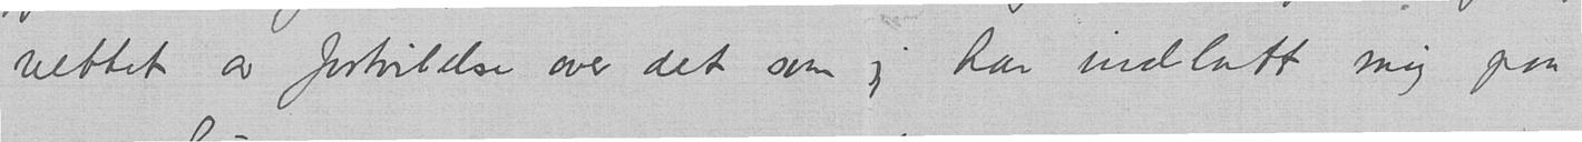vettet av fortvilelse over det som jeg har indlatt mig paa
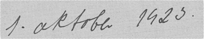1. oktober 1923.
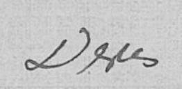Deres
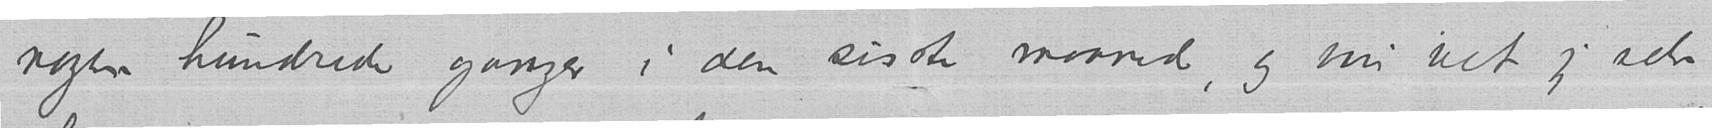nogen hundrede ganger i den sisste maaned, og nu vet jeg selv
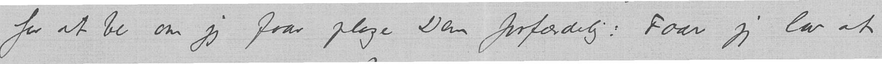for at be om jeg faar plage Dem forfærdelig: Faar jeg lov at
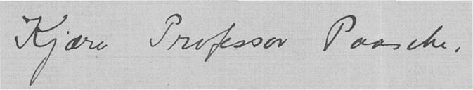Kjære Professor Paasche,
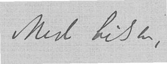Med hilsen,
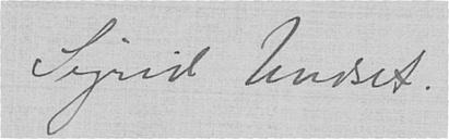Sigrid Undset.
